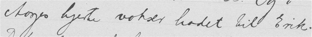Aages hjerte vokser hadet til Erik.
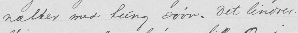nætter med tung søvn. Det lindrer.
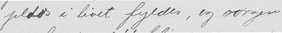plads i livet fyldes, og sorgen
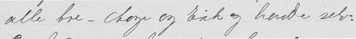alle tre – Aage og Erik og hende selv.
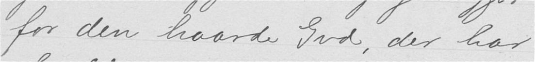for den haarde Gud, der har
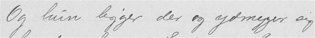Og hun ligger der og ydmyger sig
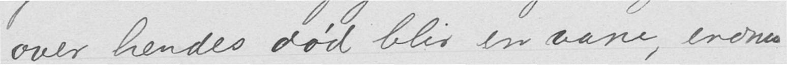over hendes død blir en vane, endnu
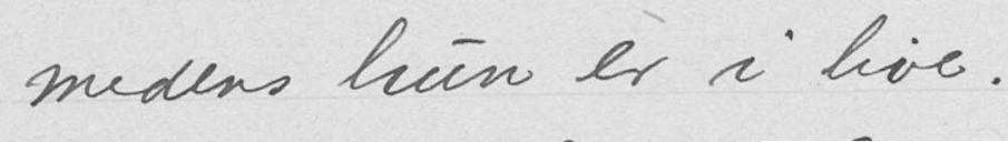medens hun er i live.
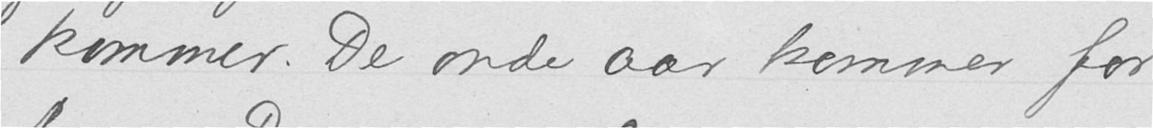kommer. De onde aar kommer for
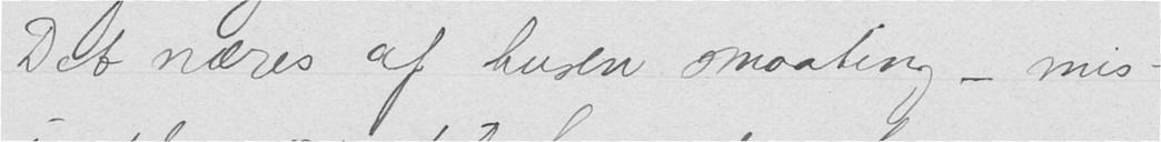Det næres af tusen smaating – mis-
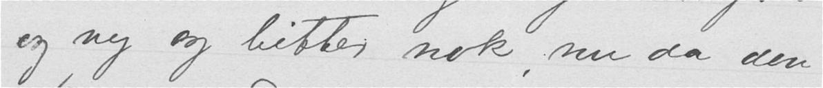og ny og bitter nok, nu da den
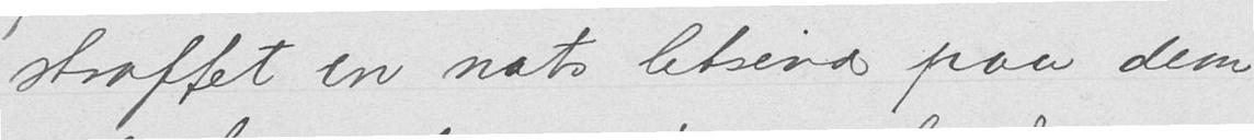straffet en nats lettsind paa dem
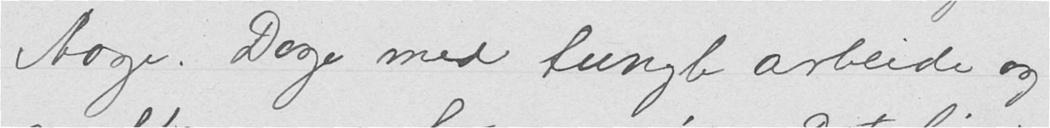Aage. Dage med tungt arbeide og
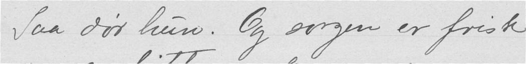Saa dør hun. Og sorgen er frisk
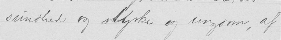sundhed og styrke og ungdom, af
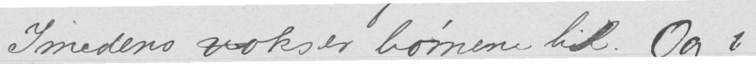Imedens vokser børnene til. Og i
